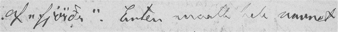af "fjörðr". Enten maatte hele navnet
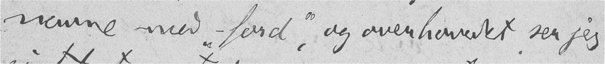navne med "-ford", og overhovedet ser jeg
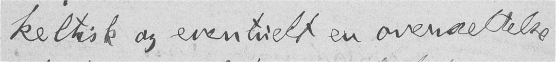keltisk og eventuelt en oversættelse
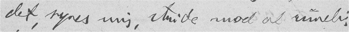det, synes mig, stride mod al rimelig-
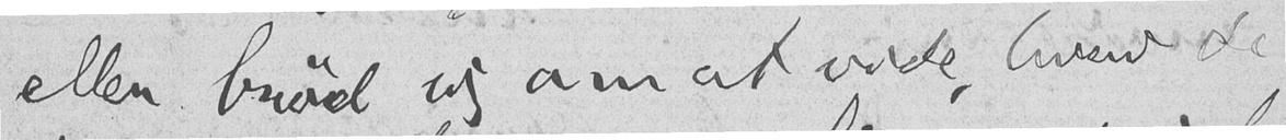eller brød sig om at vide, hvad de
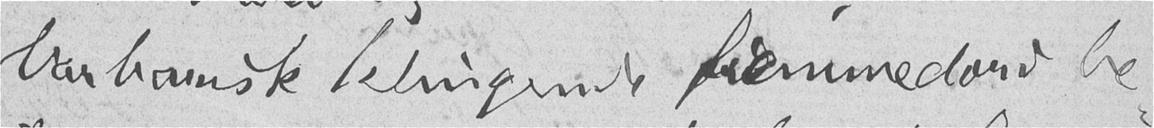barbarisk klingende fremmedord be-
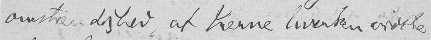omstændighed, at Irerne hverken vidste
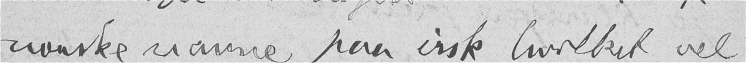norske navne paa irsk, hvilket vel
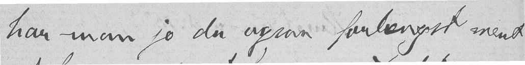har man jo da ogsaa forlængst ment
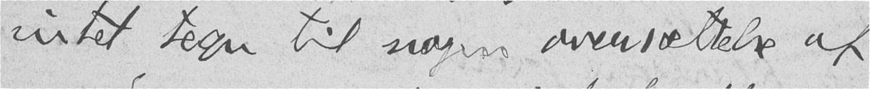intet tegn til nogen oversættelse af
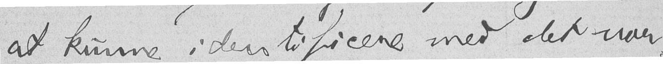at kunne identificere med det nor-
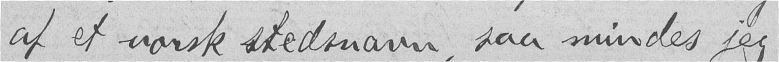af et norsk stedsnavn, saa mindes jeg
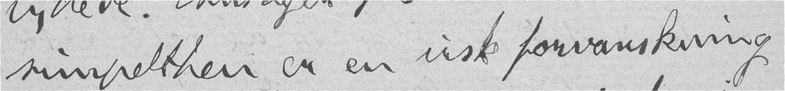simpelthen er en irsk forvanskning
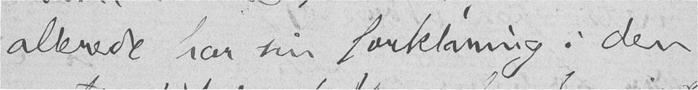allerede har sin forklaring i den
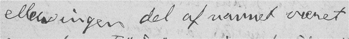eller ingen del af navnet været
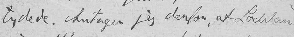tydede. Antager jeg derfor, at Lochlan
